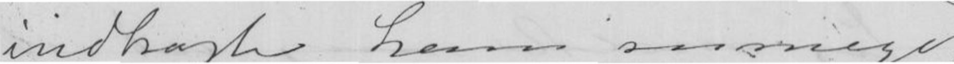indbragte han saa meget
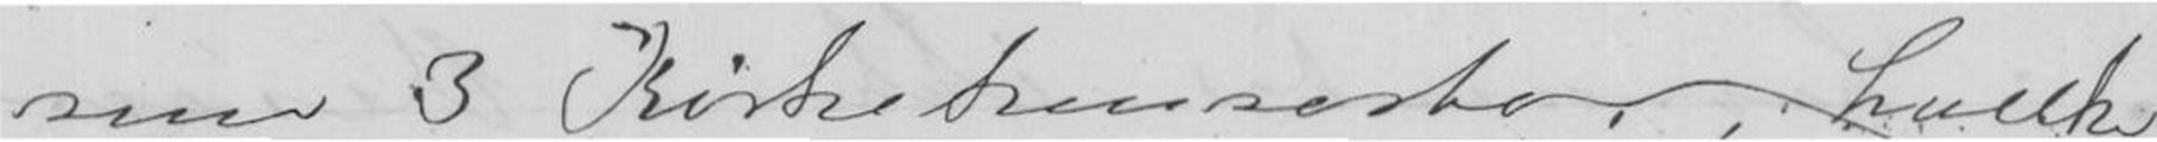sine 3 Kirkekonserter, hvilke
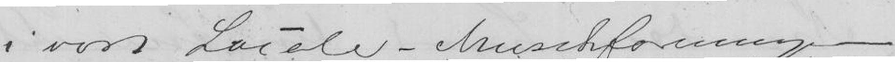i vort Locale-Musikforeninger
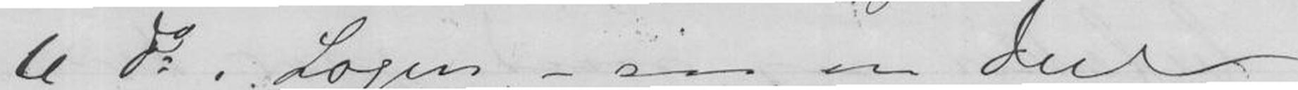6 Do. Logen. - saa en Deel
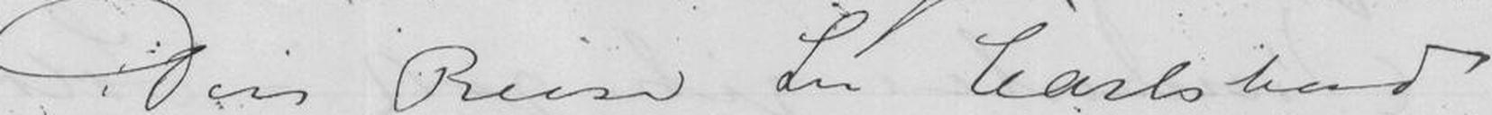Din Reise til Carlsbad
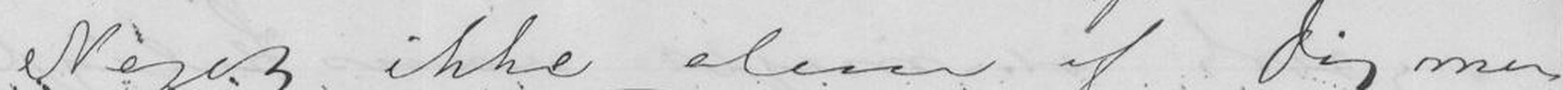Noget ikke alene af Dig men
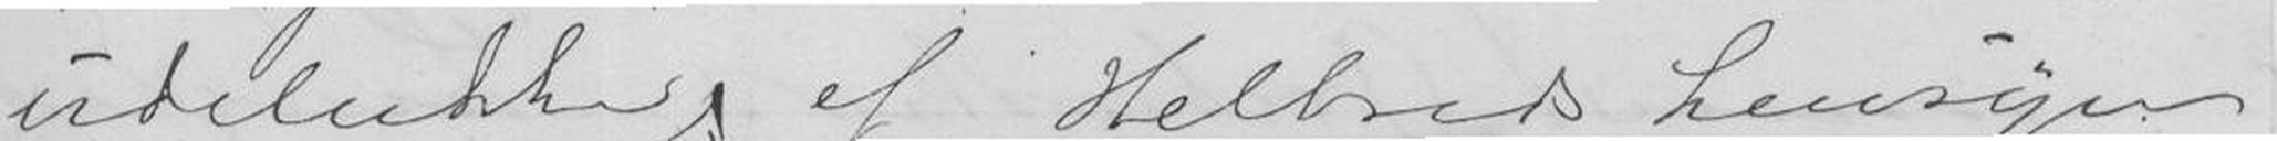udelukker har gjort af helbreds hensyn
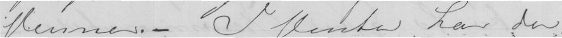Venner. - I Vinter har der
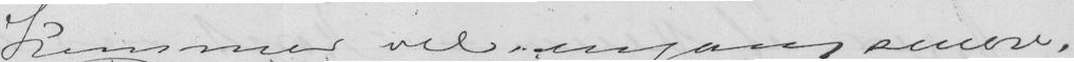kommer vel engang senere.
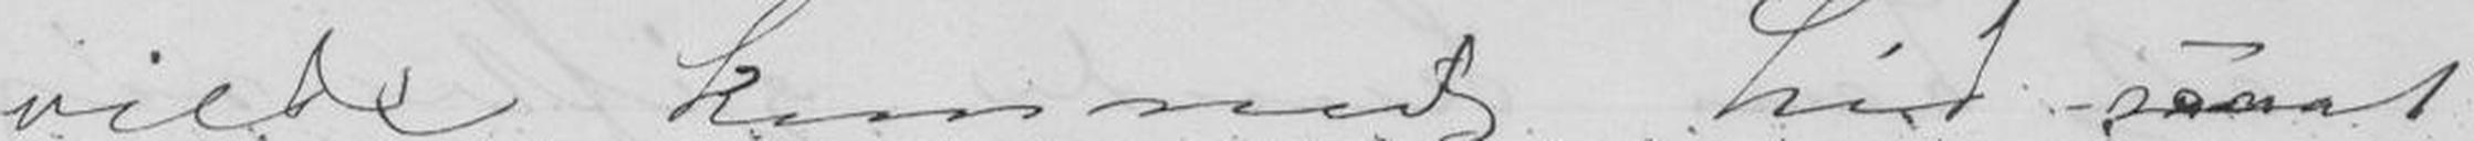vilde kommet hid saaat
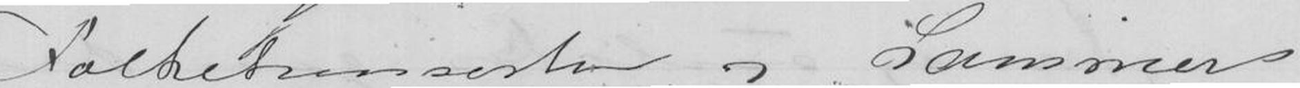Folkekonserter og Lammers
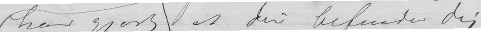at Du befinder Dig
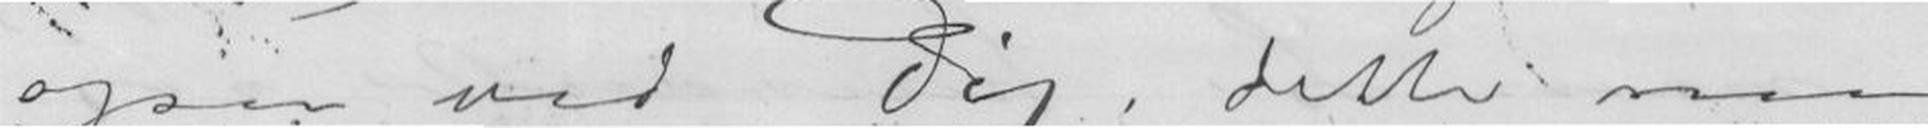ogsaa ved Dig, dette maa
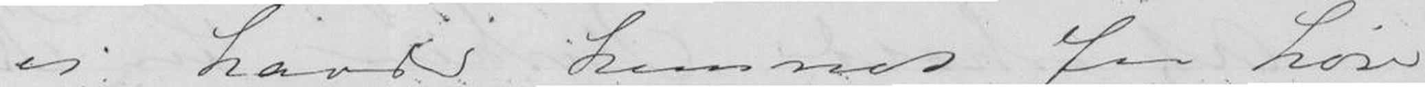vi havde kunnet faa høre
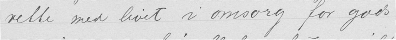rette med livet i omsorg for gods
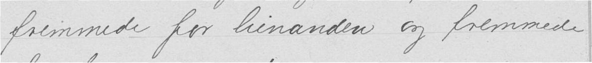fremmede for hinanden og fremmede
